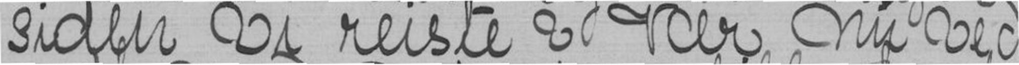siden vi reiste? Vær nu ved
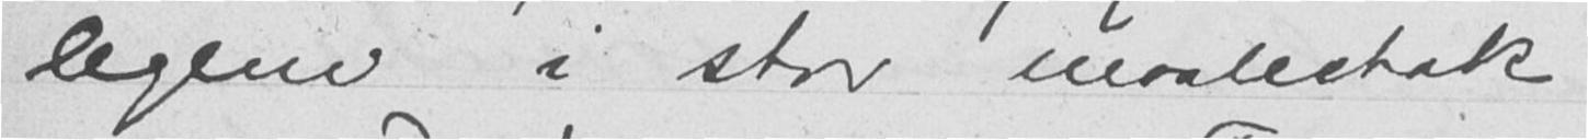legeme i stor maalestok
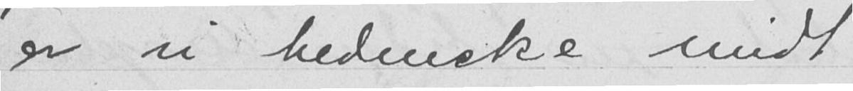er vi hedenske midt
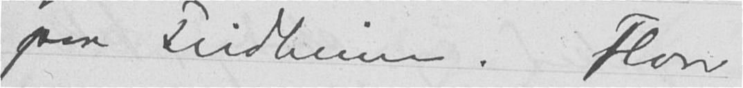paa Fridheim. Hvor
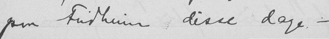paa Fridheim, disse dage -
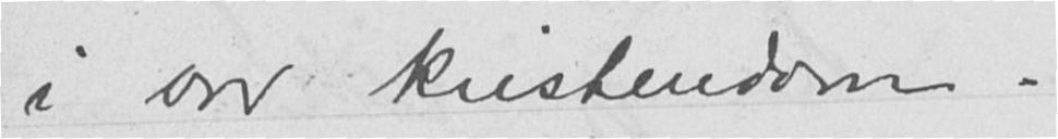i vor kristendom.
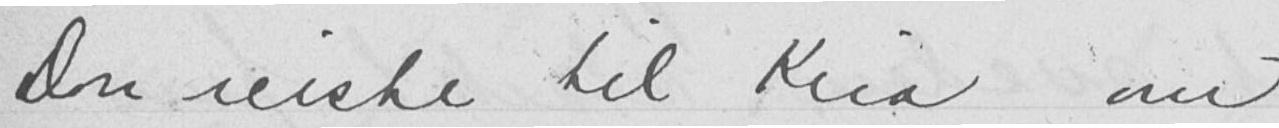Don reiste til Kria om
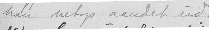han netop aandet ud.
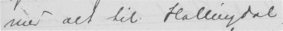med alt til Hallingdal,
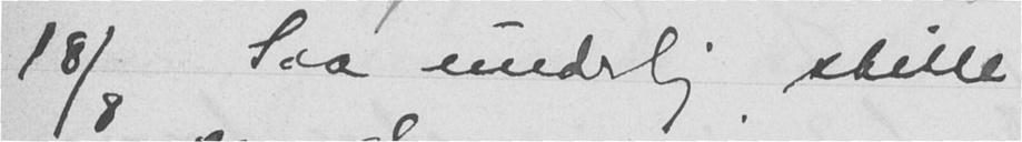18/8 Saa underlig stille
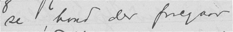se, hvad der foregaar
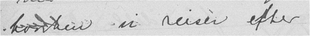hvorhen vi reiser efter
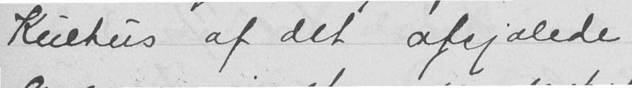Kultus af det afsjælede
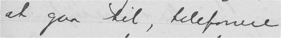at gaa til, telefonere
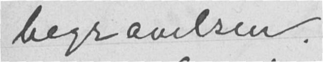begravelsen.
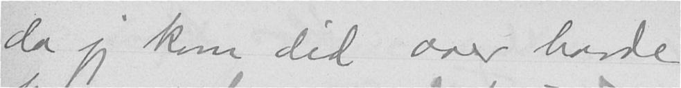da jeg kom did over havde
